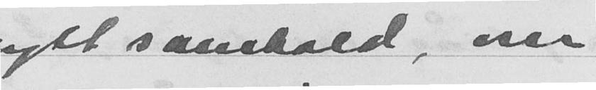snytt samhold, om
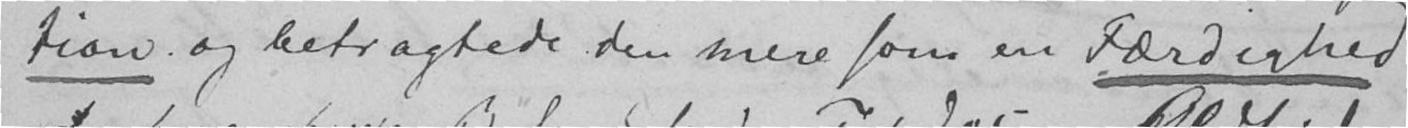tion og betragtede den mere som en Færdighed
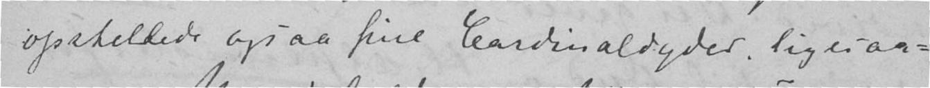opstillede ogsaa sine Cardinaldyder ligesaa-
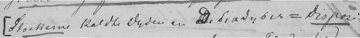Stoikerne kaldte Dyden en Diadyos – Disposi-
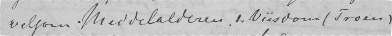velsom Middelalderen. Visdom (Troen)
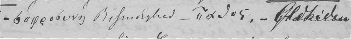– gresk Besindighed – gresk. – Oldtiden
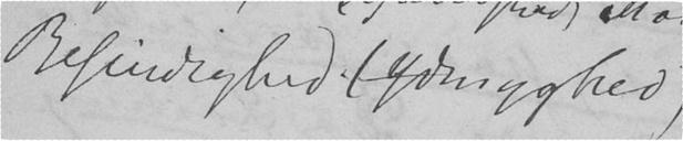Besindighed (Ydmyghed)
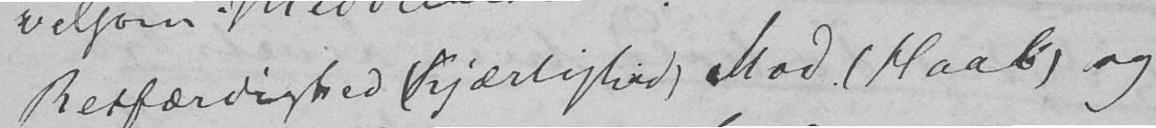Retfærdighed (Kjærlighed) Mod (Haab) og
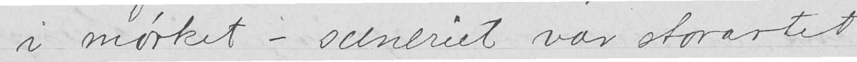i mørket - sceneriet var storartet
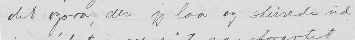det ogsaa, der jeg laa og stirrede ud
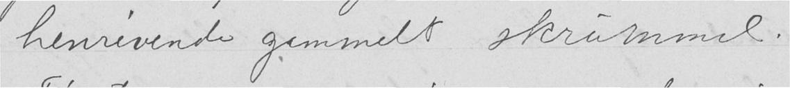henrivende gammelt skrammel.
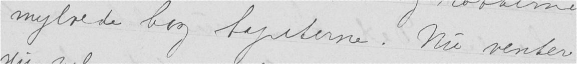myldrede bag tapeterne. Nu venter
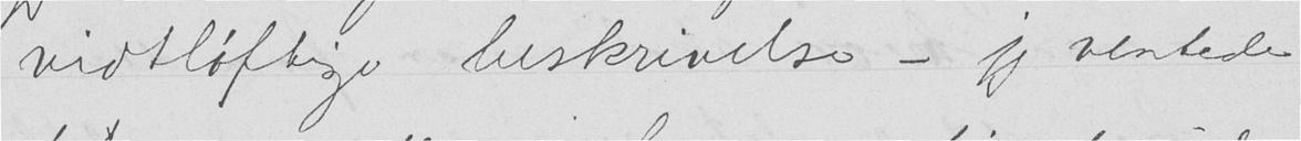vidtløftige beskrivelse - jeg ventede
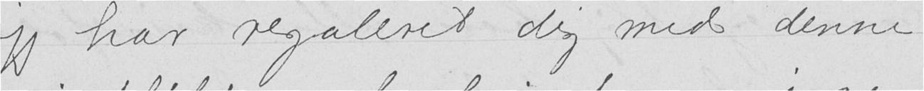jeg har regaleret dig med denne
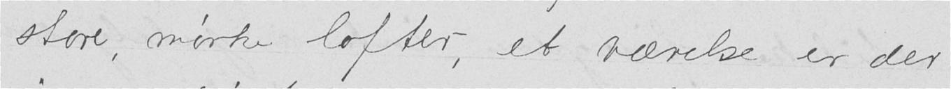store, mørke lofter, et værelse er der
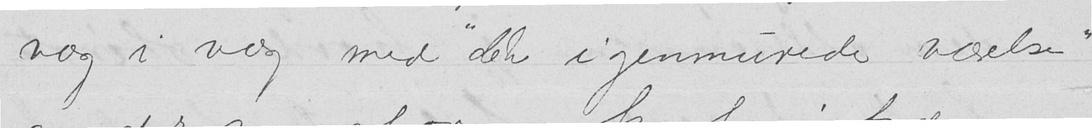væg i væg med "det igenmurede værelse"
